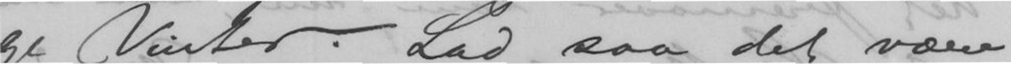ge Vinter. Lad saa det være
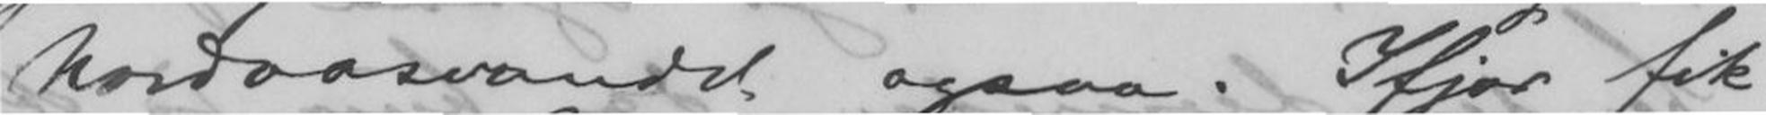Nordaasvandet ogsaa. Ifjor fik
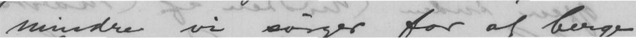mindre vi sørger for at berge
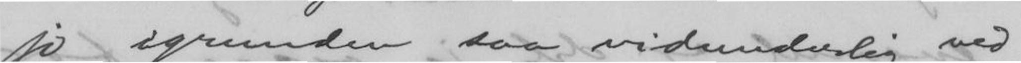jo igrunden saa vidunderlig ved
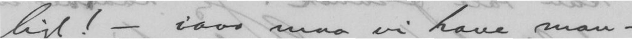ligt! - iaar maa vi have man-
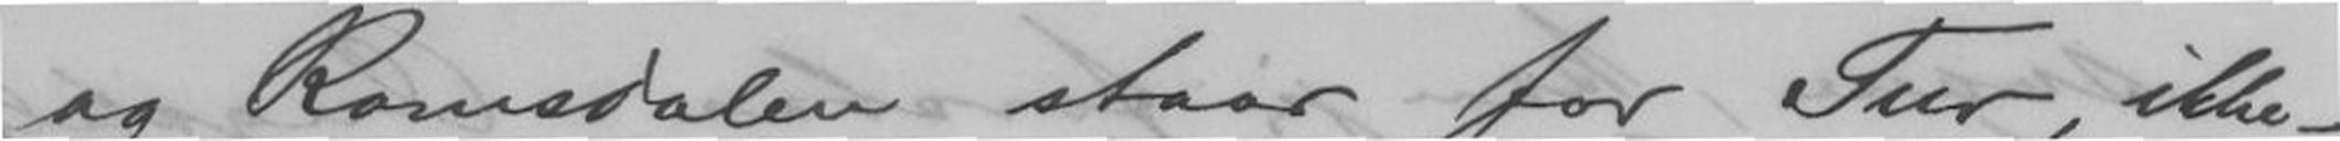og Romsdalen staar for Tur, ikke
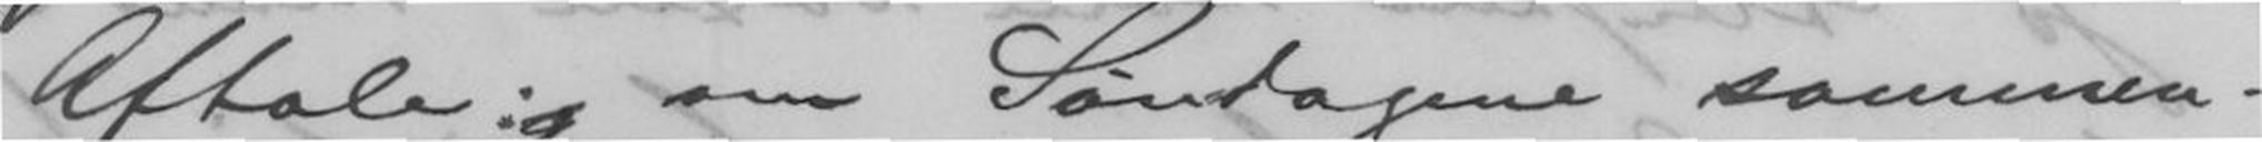Aftale: om Søndagene sammen-
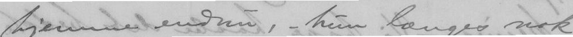hjemme endnu, - hun længes nok
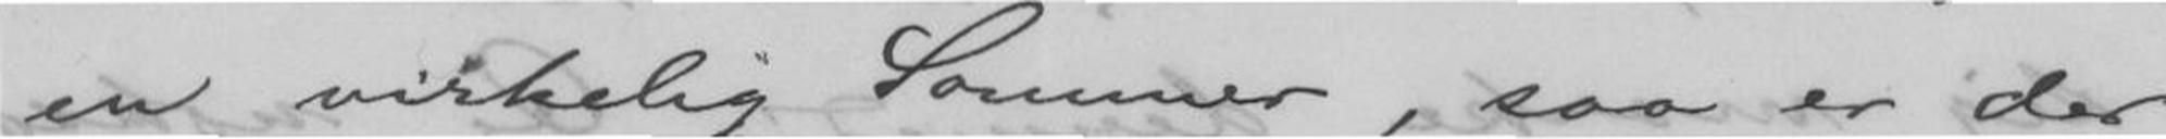en virkelig Sommer, saa er der
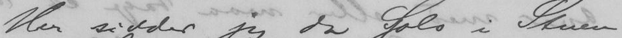Her sidder jeg da Solo i Stuen
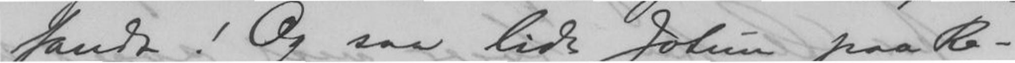sandt! Og saa lidt Jotun paa Re-
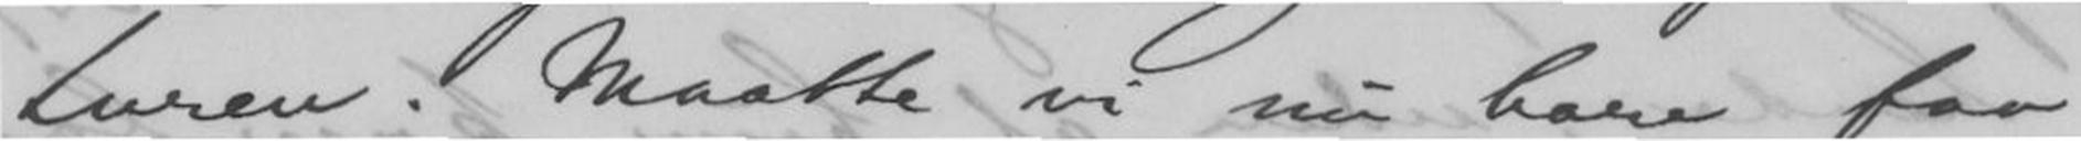turen. Maatte vi nu bare faa
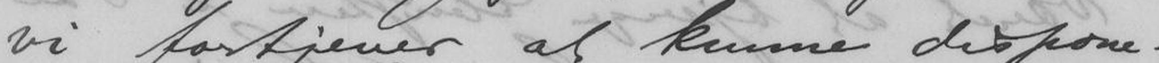vi fortjener at kunne dispone-
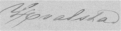Hvalstad
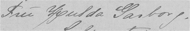Fru Hulda Garborg
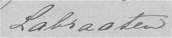Labraaten
